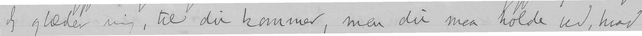Jeg glæder mig, til du kommer, men du maa holde ved, hvad
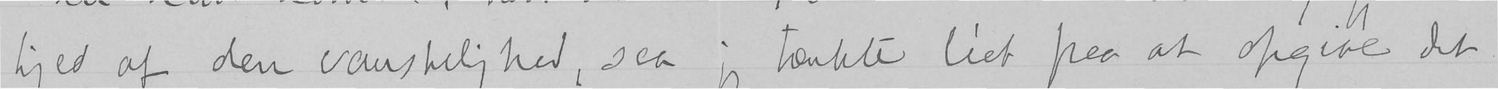kjed af den vanskelighed, saa jeg tænkte lidt paa at opgive det
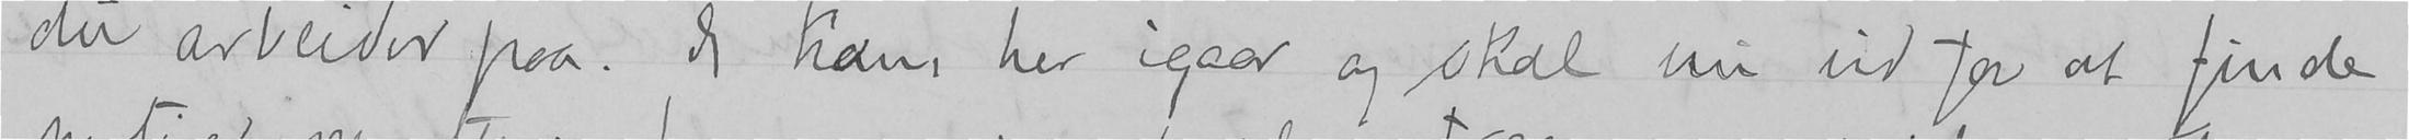du arbeider paa. Jeg kom her igaar og skal nu ud for at finde
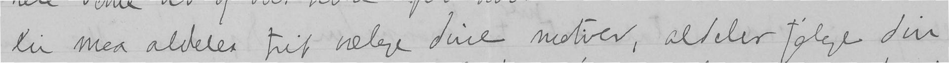Du maa aldeles frit vælge dine motiver, aldeles følge din
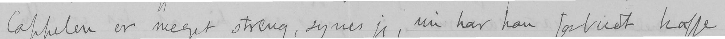Cappelen er meget streng, synes jeg, nu har han forbudt kaffe
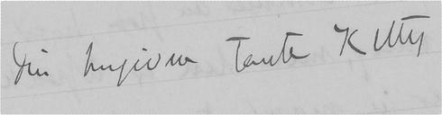Din hengivne tante Kitty.
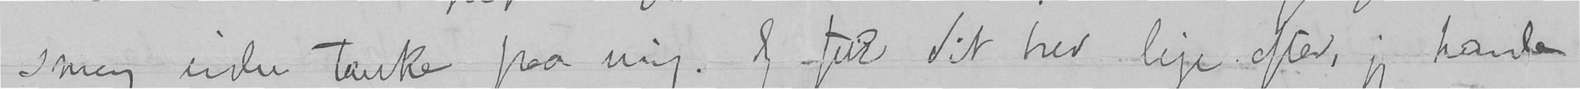smag uden tanke paa mig. Jeg fik dit brev lige efter, jeg havde
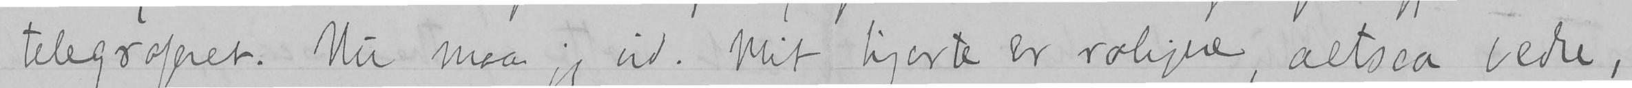telegraferet. Nu maa jeg ud. Mit hjerte er roligere, altsaa bedre,
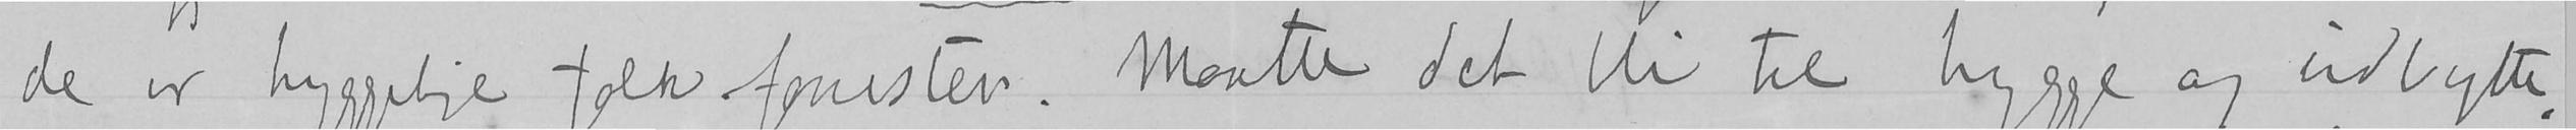de er hyggelige folk forresten. Maatte det bli til hygge og udbytte.
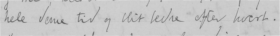hele denne tid og blit bedre efter hvert.
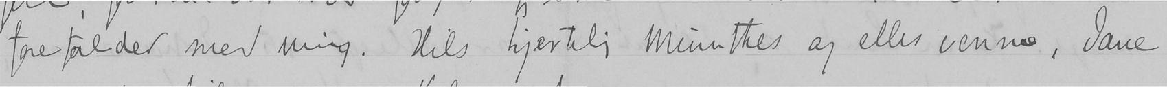forefalder med mig. Hils hjertelig Munthes og ellers venner, Jane
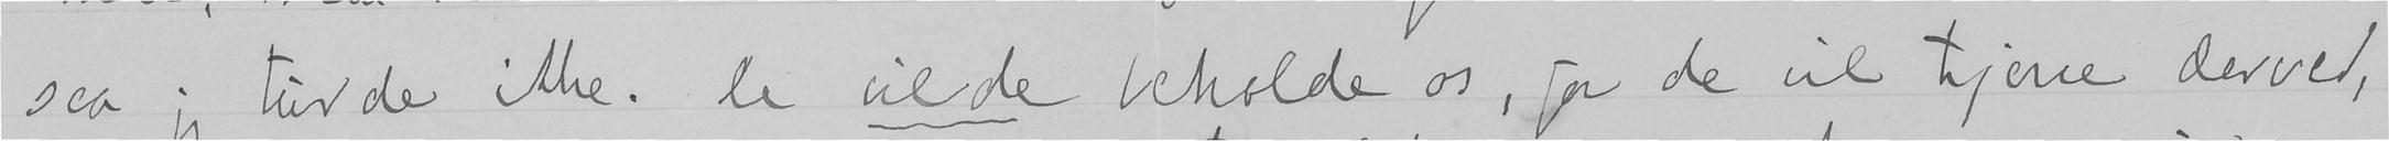saa jeg turde ikke. De vilde beholde os, for de vil tjene derved,
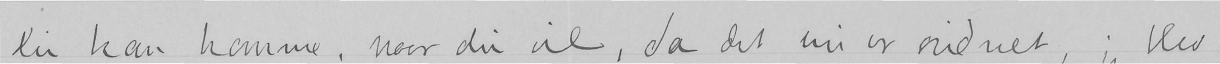Du kan komme, naar du vil, da det nu er ordnet, jeg blev
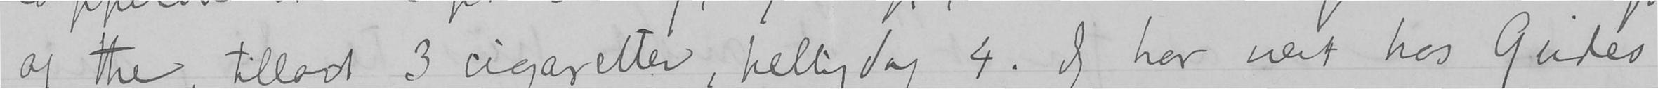og the, tilladt 3 cigaretter, helligdag 4. Jeg har vært hos Gudes
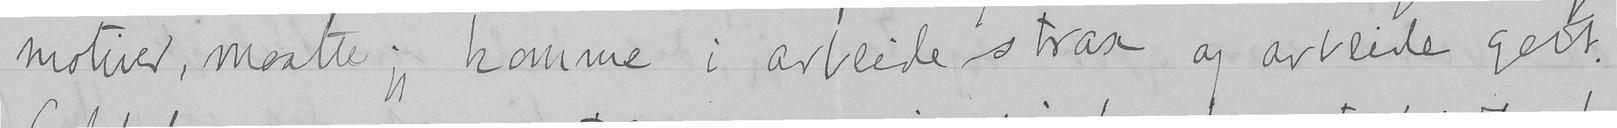motiver, maatte jeg komme i arbeide strax og arbeide godt.
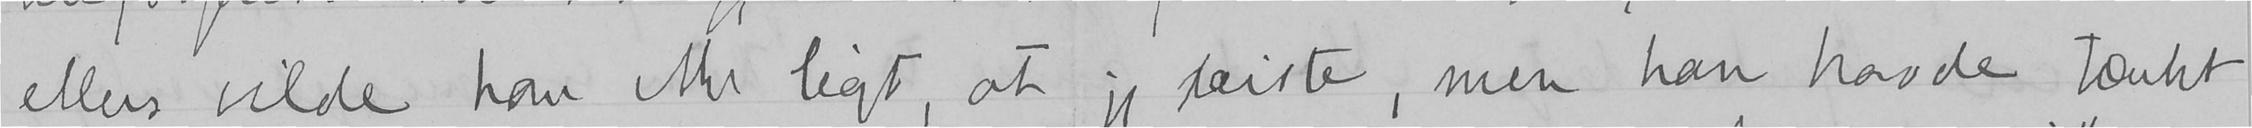ellers vilde han ikke ligt, at jeg reiste, men han havde tænkt
